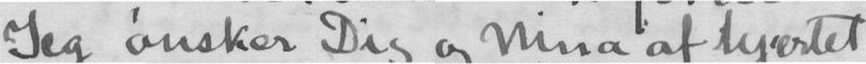Jeg ønsker Dig og Nina af hjertet
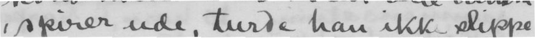spirer ude, turde han ikke slippe
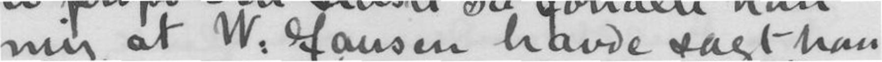mig at W. Hansen havde sagt han
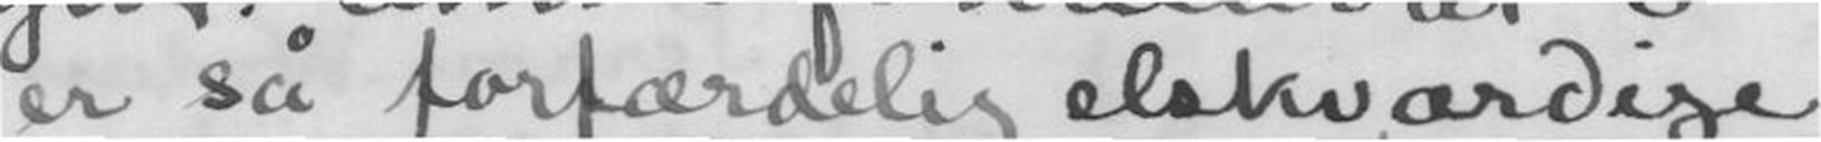er så forfærdelig elskværdige
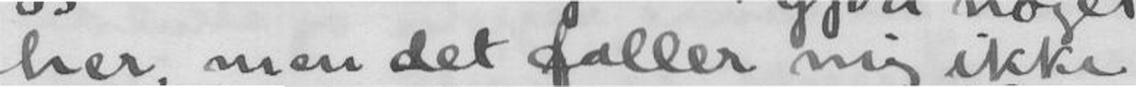her, men det faller mig ikke
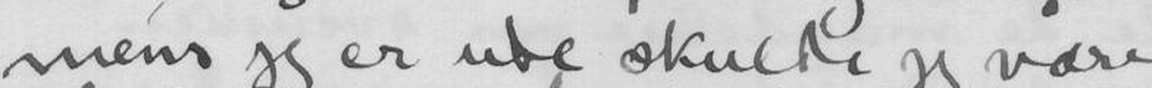mens jeg er ute skulde jeg være
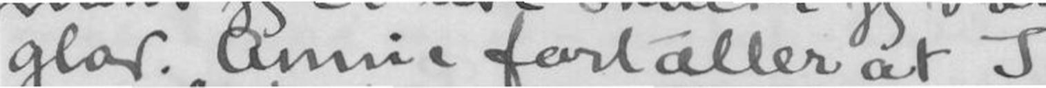glad. Annie fortæller at I
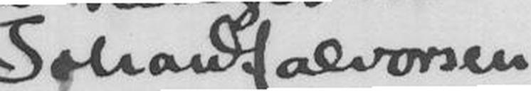Johan Halvorsen
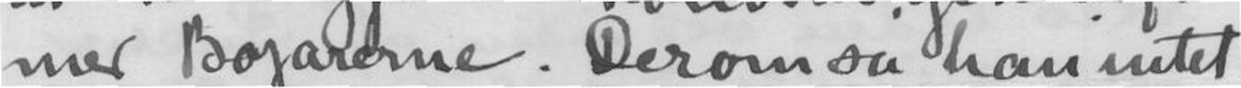med Bojarerne. Derom sa han intet
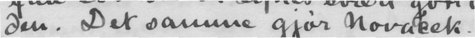den. Det samme gjør Novacek.
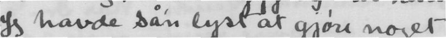Jeg havde så'n lyst at gjøre noget
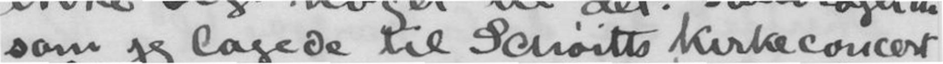som jeg lagede til Schøitts kirkeconcert
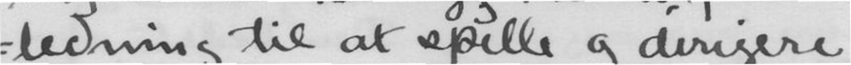ledning til at spille og dirigere
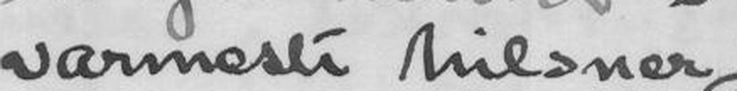varmeste hilsner.
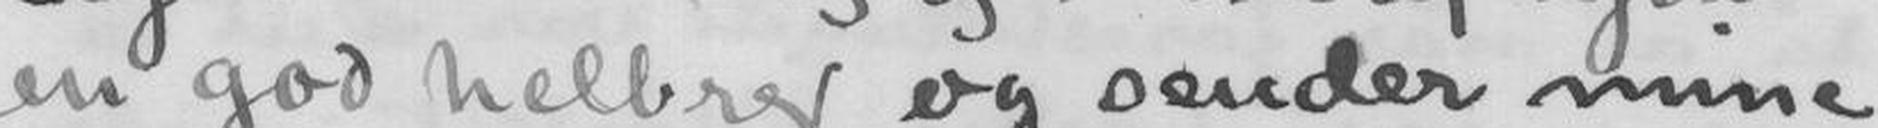en god helbred og sender mine
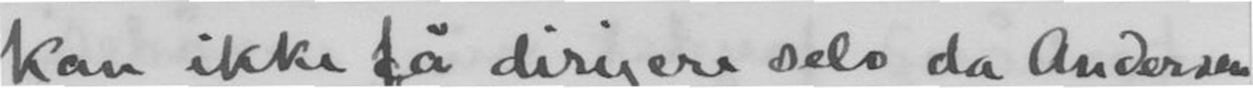kan ikke få dirigere selv da Andersen
